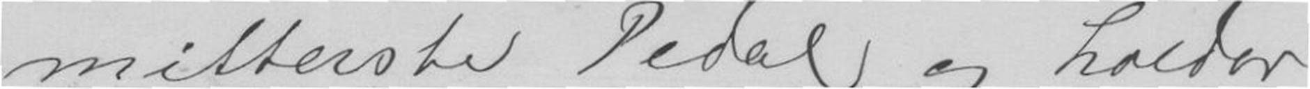mitterste Pedal og holder
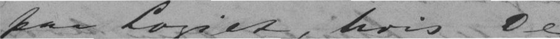paa Logiet hvis De
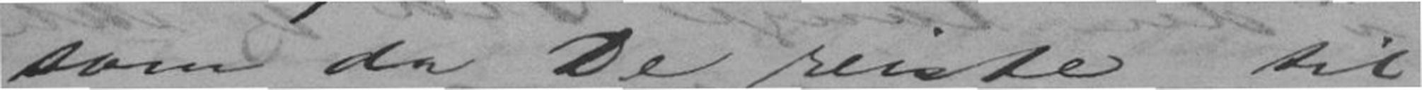som da De reiste til
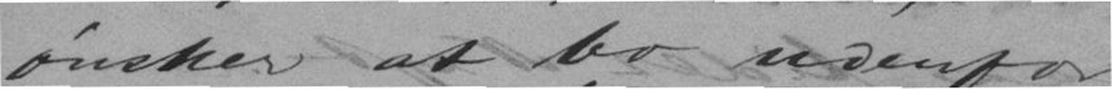ønsker at bo udenfor
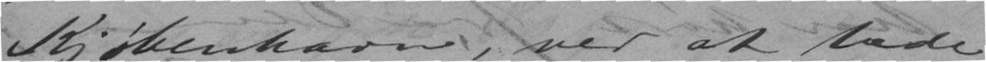Kjøbenhavn, ved at lade
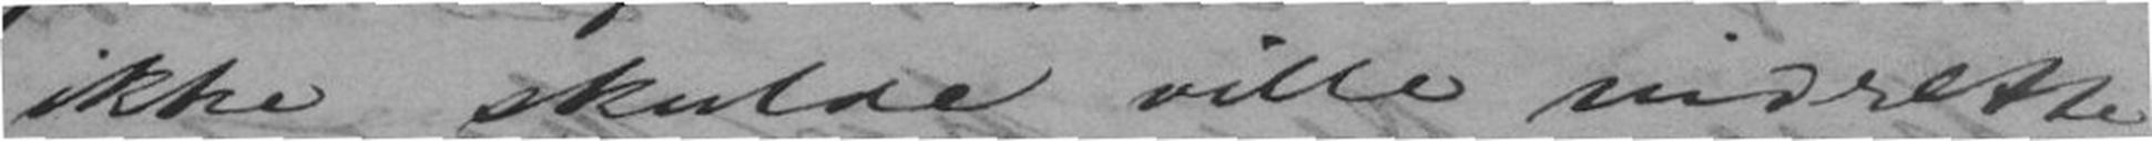ikke skulde ville indrette
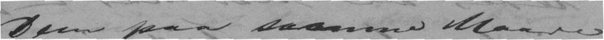Dem paa samme Maade
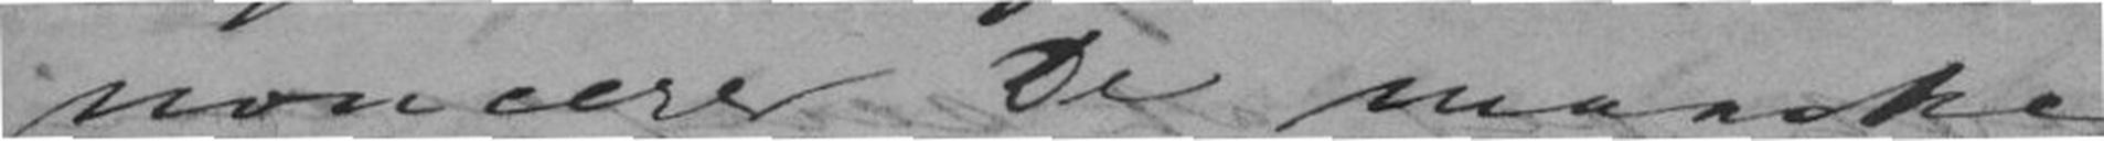noncerer De maaske
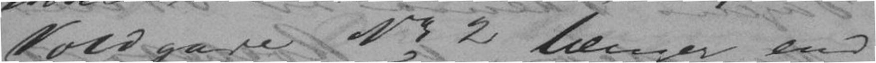Vordgade No2 længer end
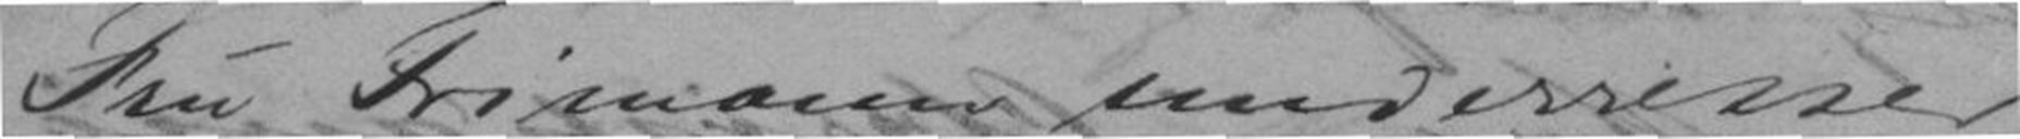Fru Frimann underretted
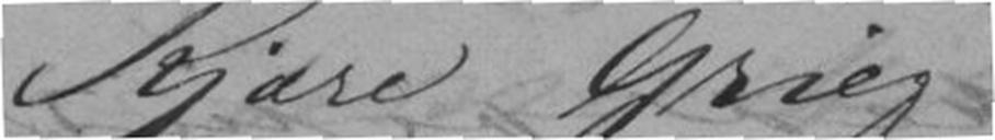Kjære Grieg!
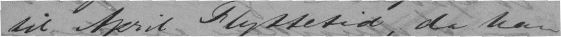til April Flyttetid, da han
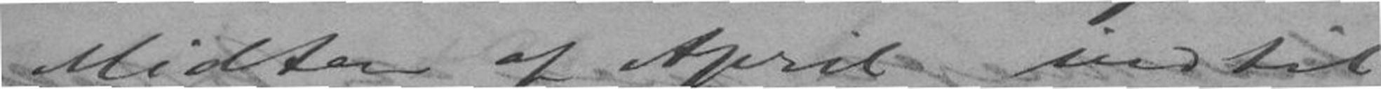Midten af April indtil
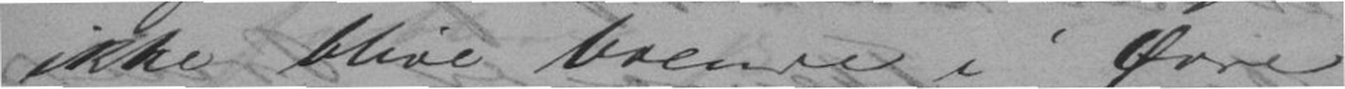ikke blive blende i Øvre
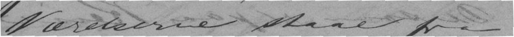Værelserne staae fra
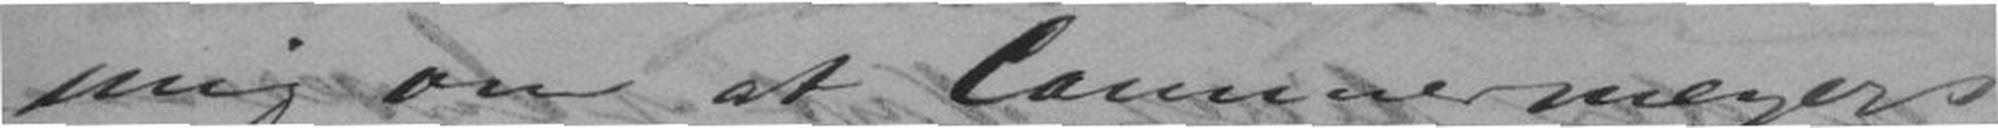mig om at Cammermeyers
